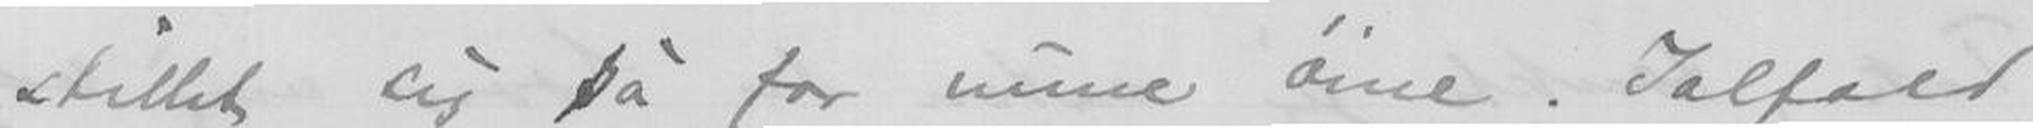stillet sig så for mine øiene. Ialfald
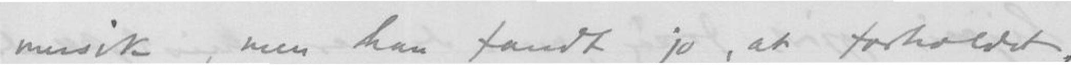musik, men han fandt jo, at forholdet
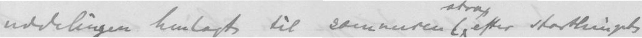uddelingen henlagt til sommeres (strax efter storthingets
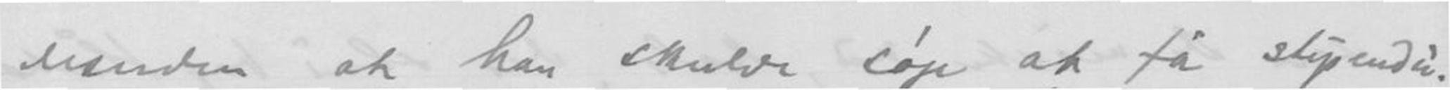desuden, at han skulde søge at få stipendie-
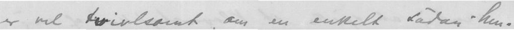er vel tvivlsomt, om en enkelt sådan hen-
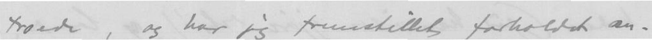troede, og har jeg fremstillet forholdet an-
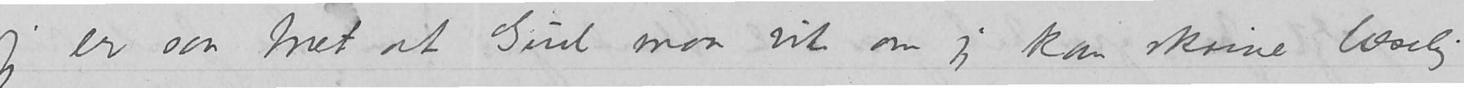jeg er saa træt at Gud maa vite om jeg kan skrive læselig
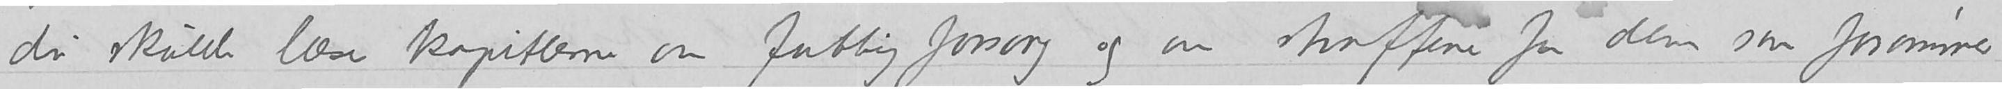du skulde læse kapitlerne om fattigforsorg og om straffene for dem som forsømmer
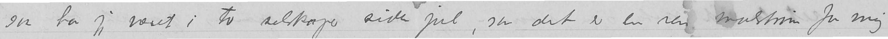saa har jeg været i to selskaper siden jul, saa det er en ren malstrøm for mig
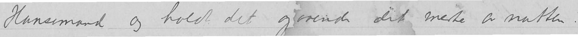Hansemand og holdt det gaaende det meste av natten.
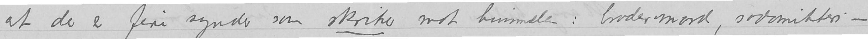at der er fire synder som skriker mot himmelen: brodermord, sodomitteri -
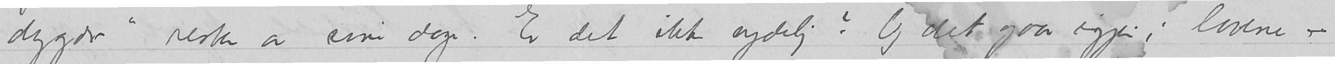dygdr» resten av sine dager. Er det ikke nydelig? Og det gaar igjen i lovene-
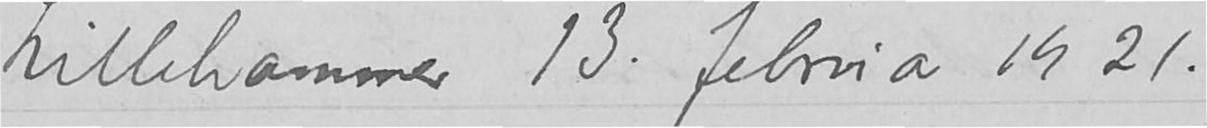Lillehammer 13. februar 1921.
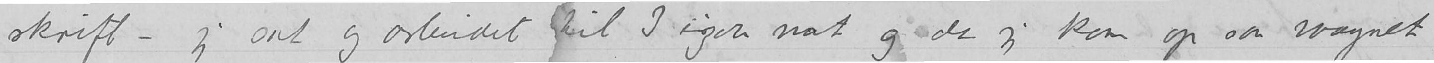skrift – jeg sat og arbeidet til 3 igaar nat og da jeg kom op saa vaagnet
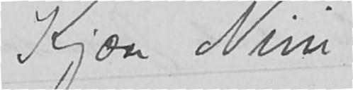Kjære Nini
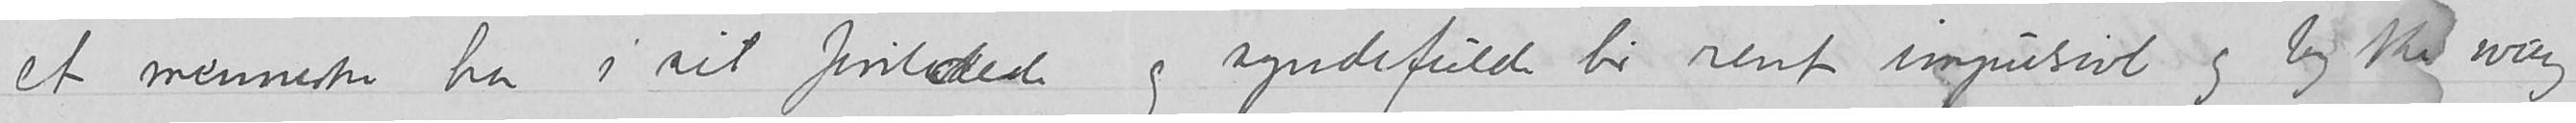et menneske har i sit forvildede og syndefulle liv rent impulsivt og by the way
